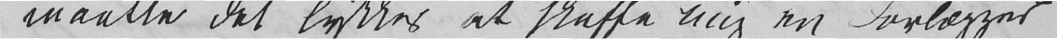maatte det lykkes at skaffe mig en Forlægger
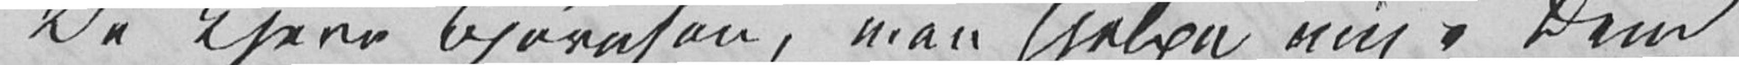De kjere Bjørnson, maa hjelpe mig. Dem
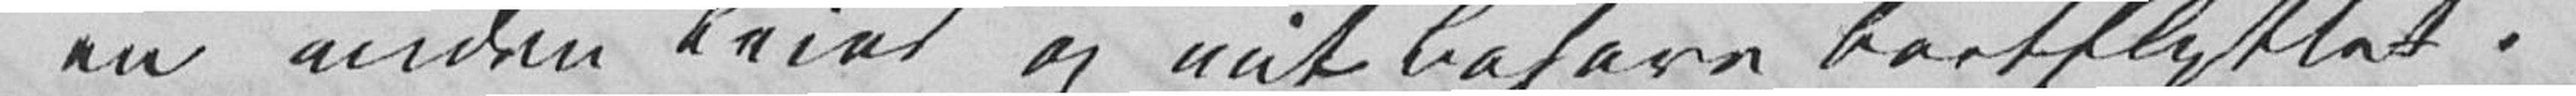en anden Leier og mit Bohave bortflyttet.
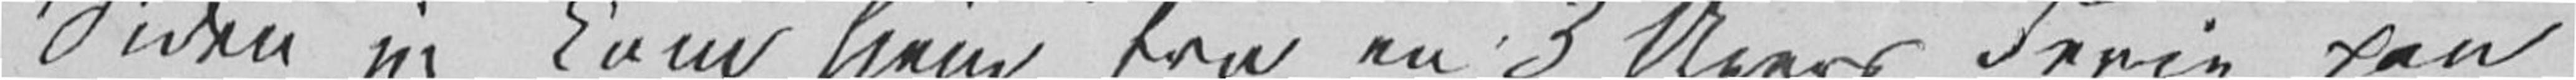Siden jeg kom hjem fra en 3 Ugers Ferie paa
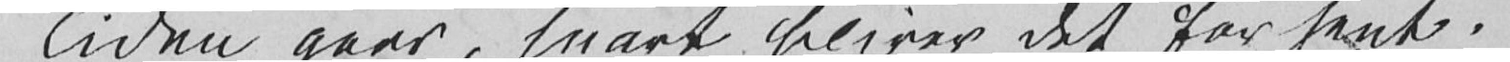Tiden gaar, snart bliver det for sent.
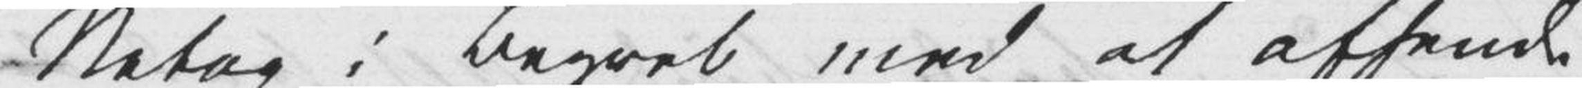Netop i Begreb med at avsende
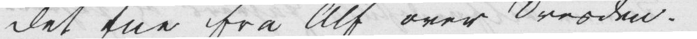Det Ene fra Alf over Dresden.
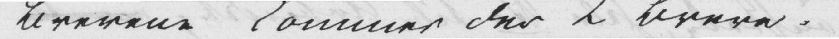Brevene kommer der 2 Breve.
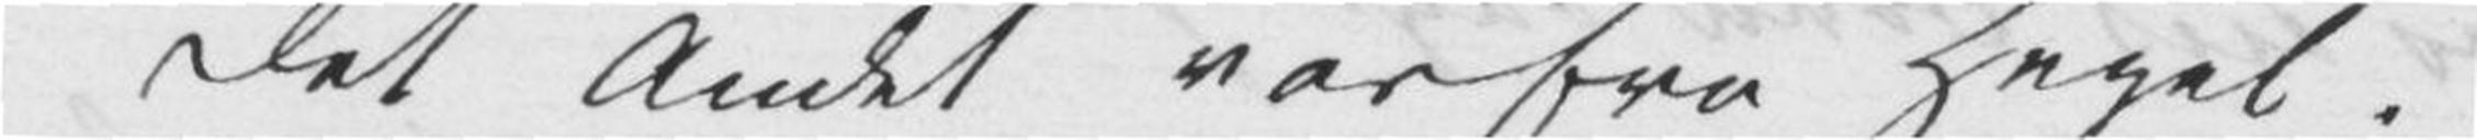Det Andet var fra Hegel.
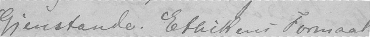Gjenstande. Ethikens Formaal
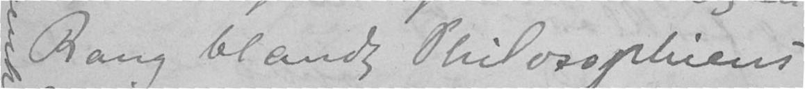Rang blandt Philosophiens
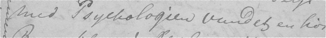med Psychologien vundet en høi
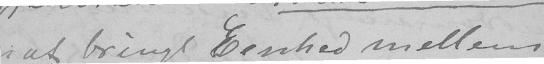er at bringe Eenhed mellem
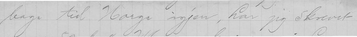bage til Norge igjen, har jeg skrevet
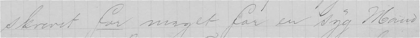skrevet for meget for en syg Mand
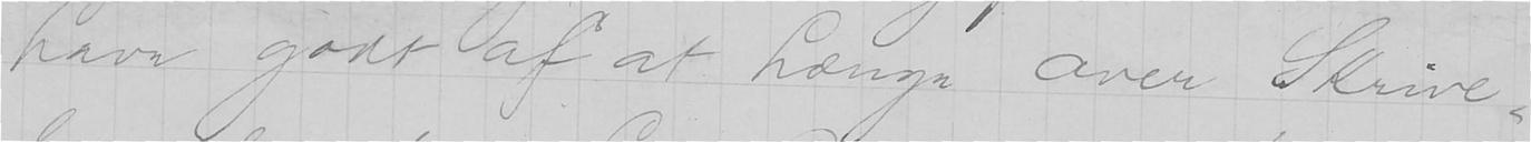have godt af at hænge over Skrive-
